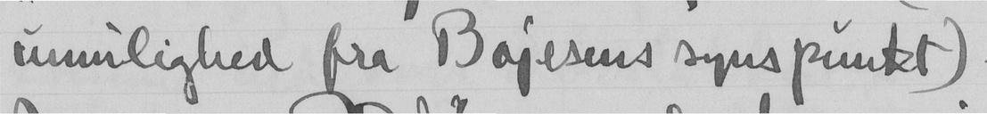umulighed fra Bojesens synspunkt)
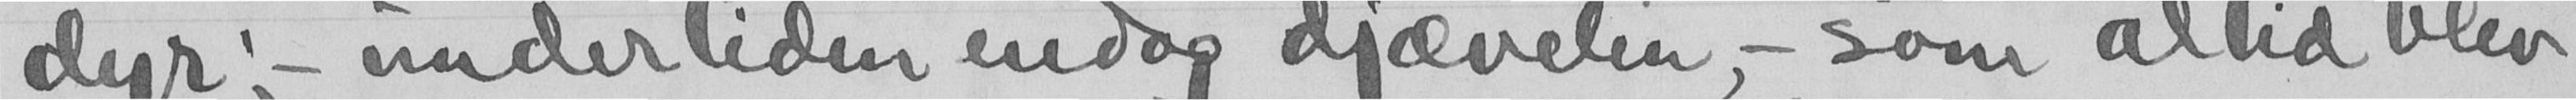dyr; - undertiden endog djævelen - som altid blev
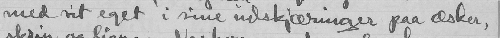med sit eget i sine udskjæriger paa æsker,
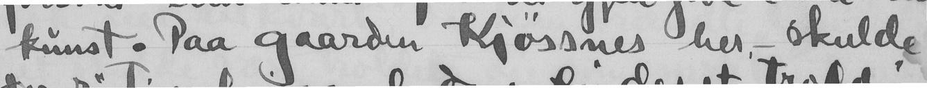kunst. Paa gaarden Kjøssnes her,- skulde
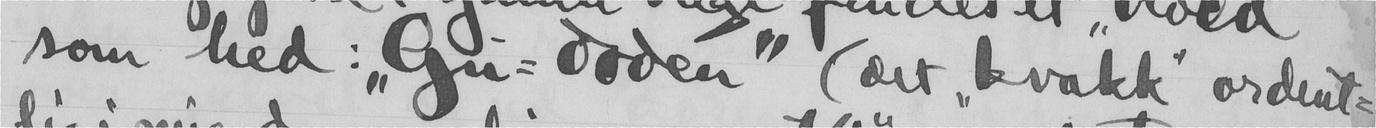som hed: "Gu-doden" (det "kvakk" ordent-
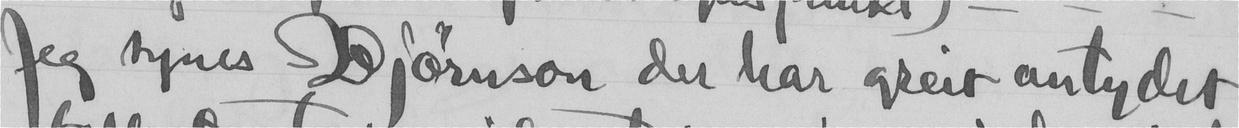Jeg synes Bjørnson der har greit antydet
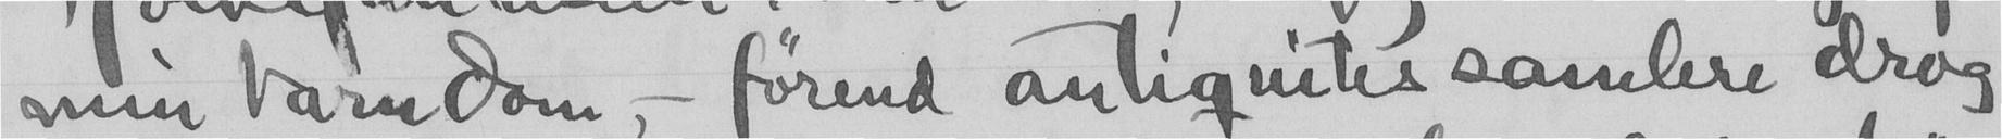min barndom, - førend antiquites samlere drog
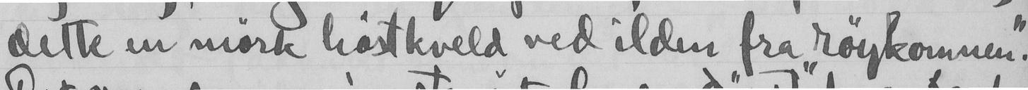dette en mørk høstkveld ved ilden fra røykommen".
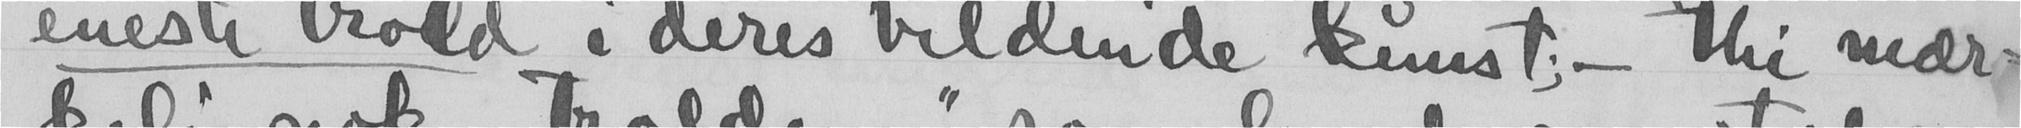eneste trold i deres bildende kunst - thi mær-
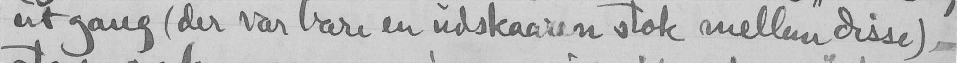utgang (der var bare en udskaaren stok mellem disse) -
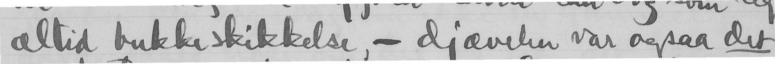altid bukkeskikkelse - djævelen var ogsaa det
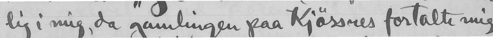lig i mig, da gamlingen paa Kjøssnes fortalte mig
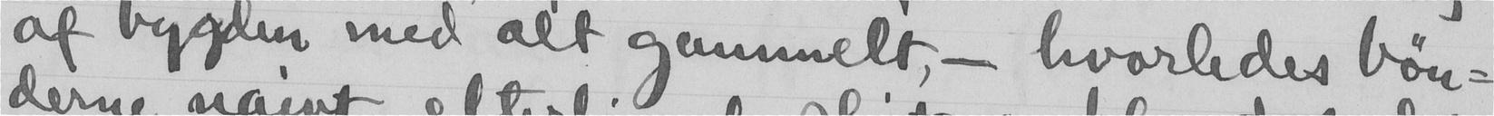af bygden med alt gammelt, - hvorledes bøn-
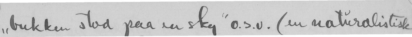"bukken stod paa en sky" o.s.v. (en naturalistisk
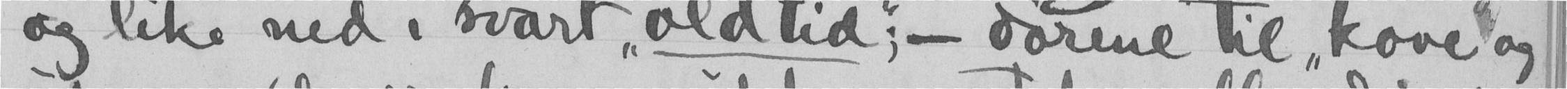og like ned i svart "oldtid"; - dørene til "kove" og
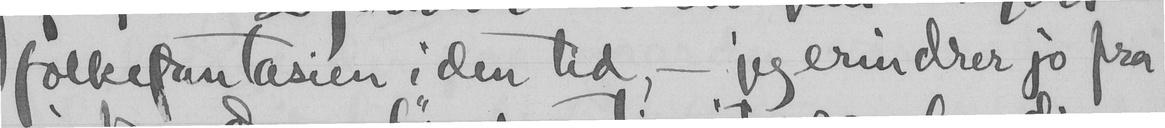folkefantasien i den tid, - jeg erindrer jo fra
